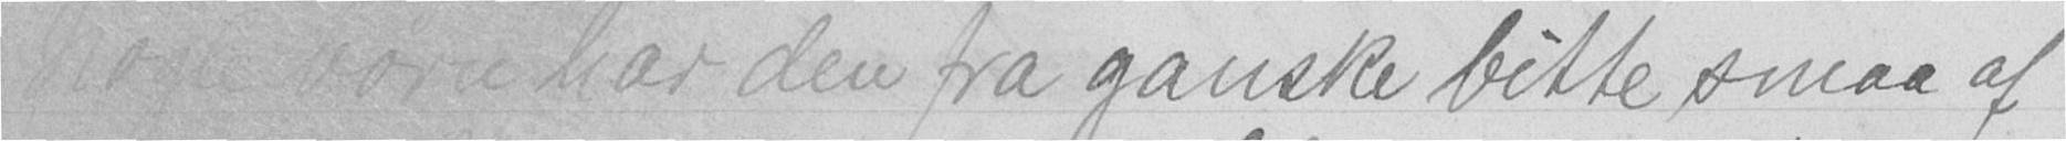Nogle børn har den fra ganske bitte smaa af
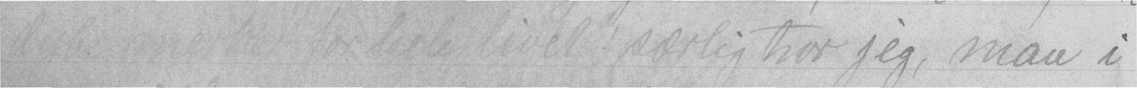dybe merker for hel livet! særlig tror jeg, man i
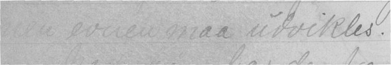men evnen maa udvikles.
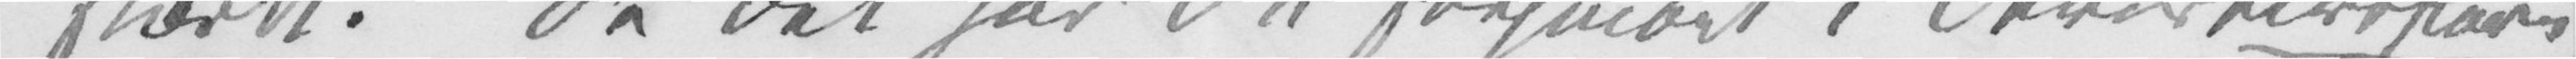stærkt. Se det har De forsmået i Deres «Livsslave»
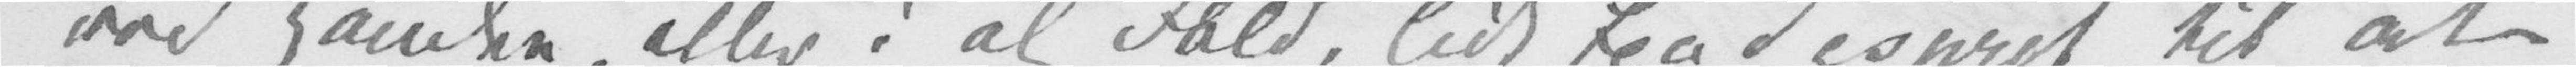ved Hånden, eller i al Fald, lidt Eau d'esprit til at
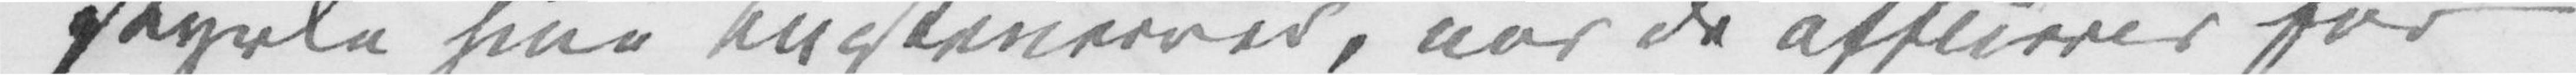styrle sine Lugtenerver, når de afficeres for
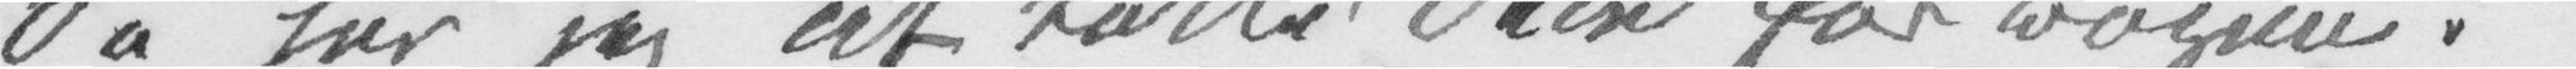Så har jeg at takke Dem for Bogen.
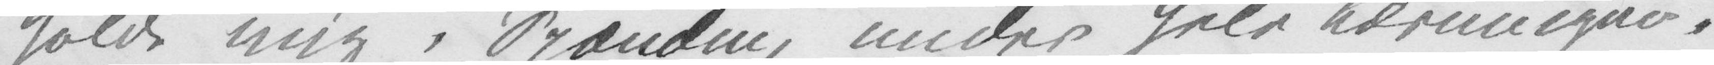holde mig i Spænding under hele Læsningen.
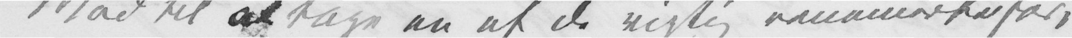Mod til at tage en af de rigtig rammeste for,
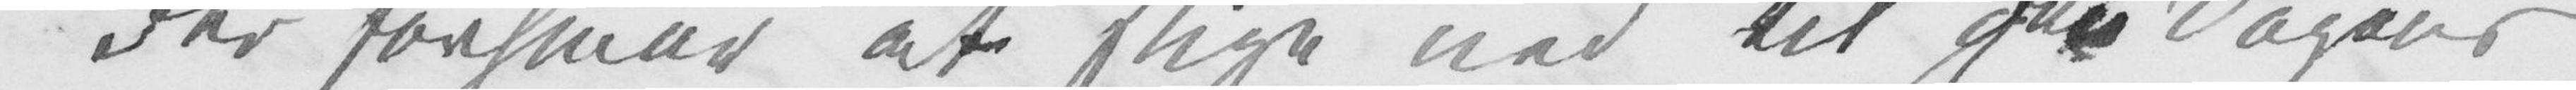der forsmår at stige ned til hin Dagens
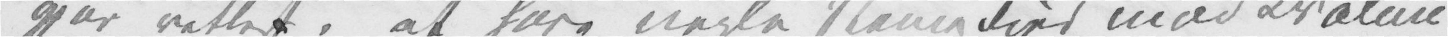gjør rettest i at have nogle Remedier mod Kvalme
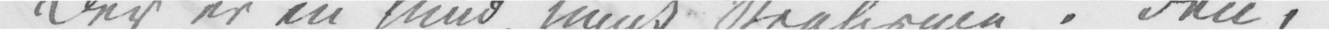Der er en sund, smuk Realisme i den,
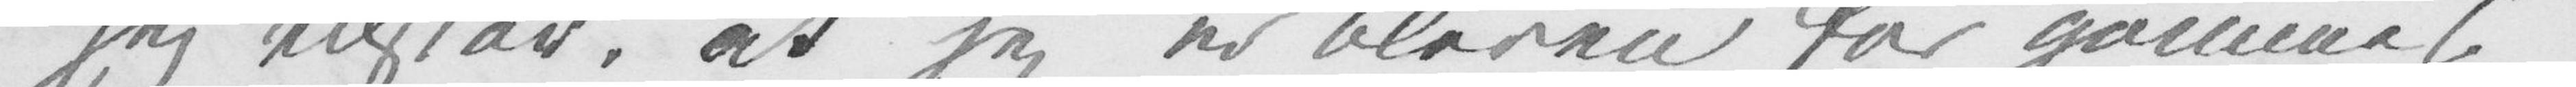Jeg tilstår, at jeg er bleven for gammel
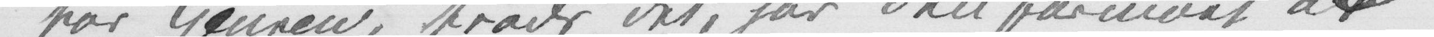for Genren, trods det, har den formået at
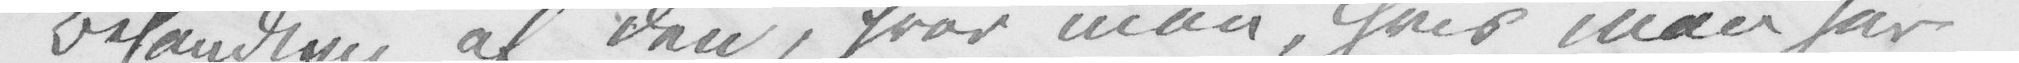Behandling af den, hvor man, hvis man har
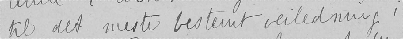til det meste bestemt veiledning i
